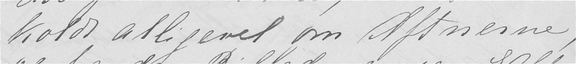Koldt alligevel om Aftnerne,
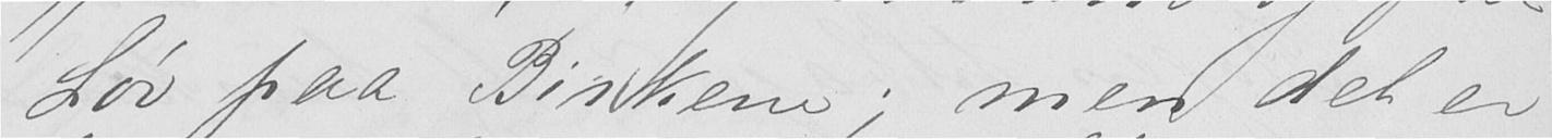Løv paa Birkene; men det er
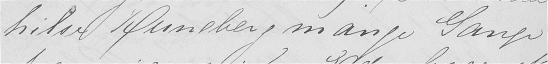hilse Runeberg mange Gange
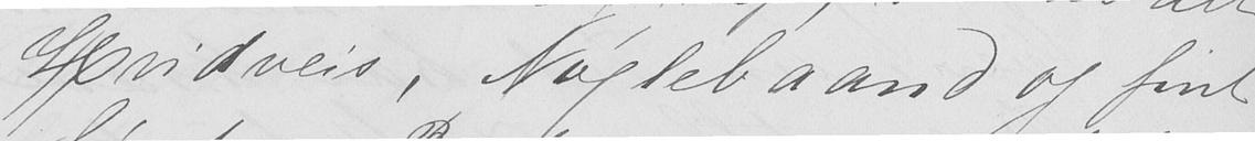Hvidveis, Nøglebaand og fint
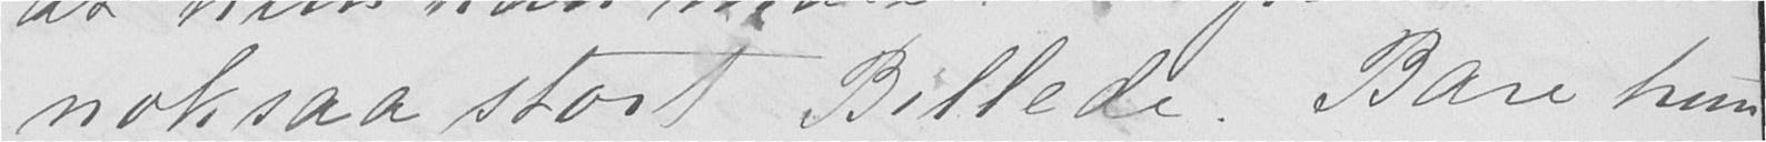noksaa stort Billede. Bare hun
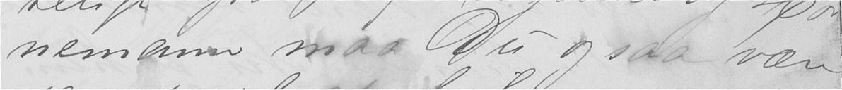nemann maa Du ogsaa være
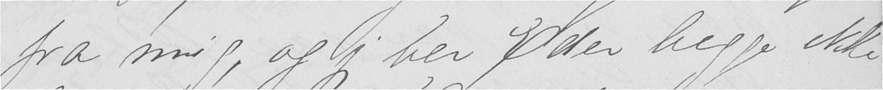fra mig, og jeg ber Eder begge ikke
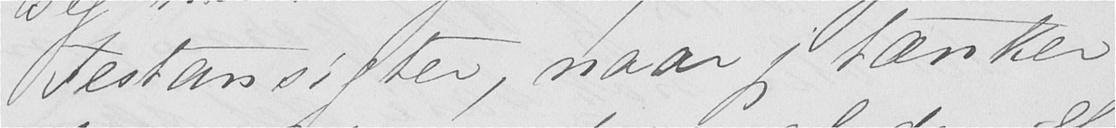Festansigter, naar jeg tænker
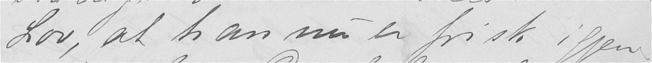Lov, at han nu er frisk igjen.
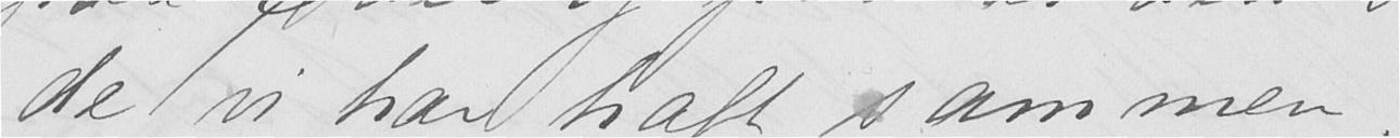de vi har haft sammen.
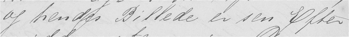og hendes Billede er sen Efter-
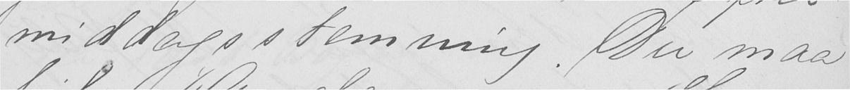middagsstemning. Du maa
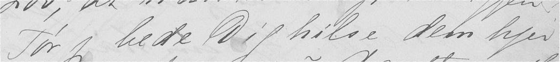Tør jeg bede Dig hilse dem hjer-
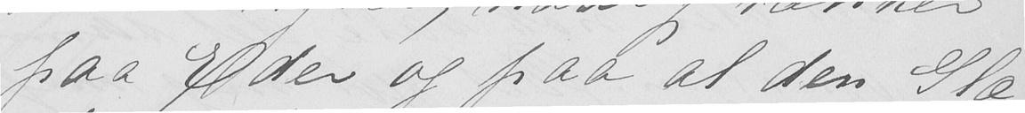paa Eder og paa al den Glæ-
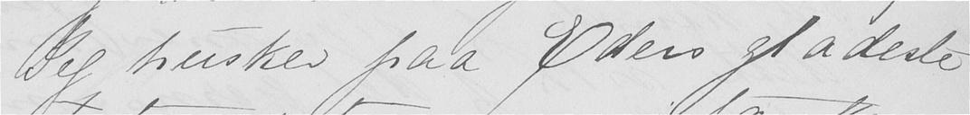Jeg husker paa Eders gladeste
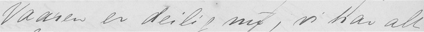Vaaren er deilig nu, vi har alt
